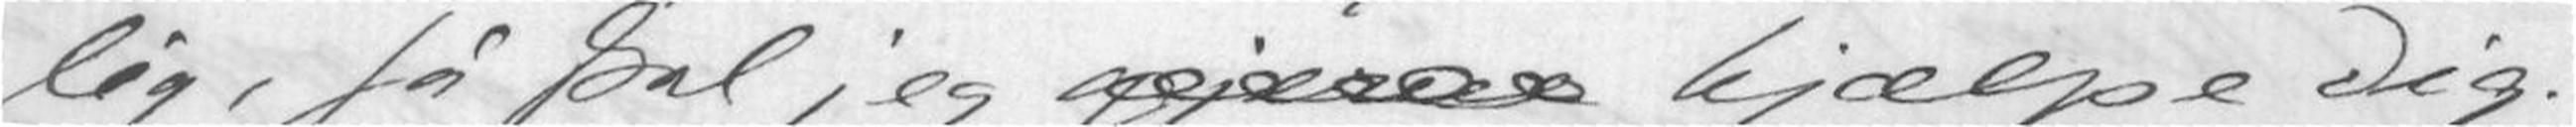lig, så skal jeg gjærne hjælpe dig.
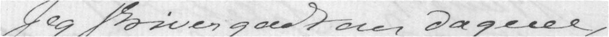Jeg skriver godt om dagene,
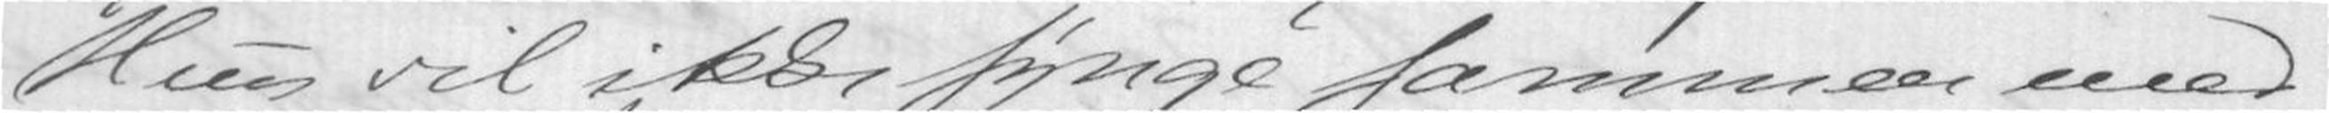Hun vil ikke synge sammen med
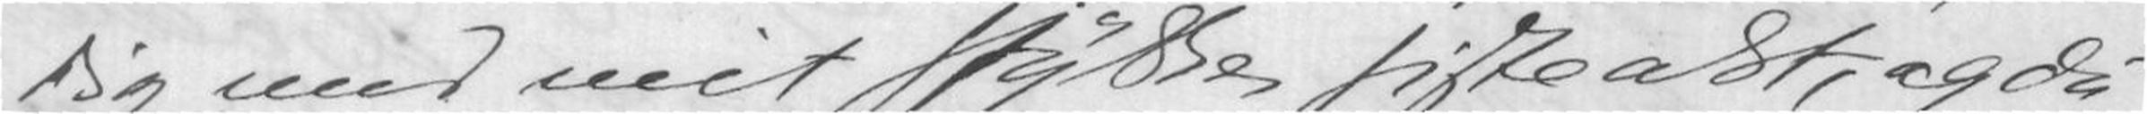dig med mit stykkes siste akt, og du
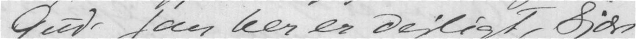Gud som her er dejligt, kjære
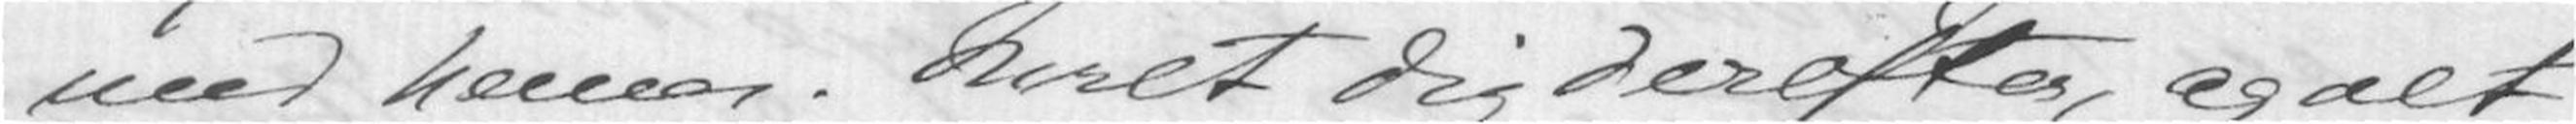med henne. Inret dig derefter, og alt
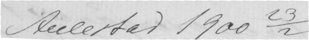Aulestad 1900 23/2
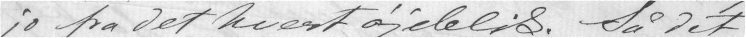jo fra det hvert øjeblik. Så det
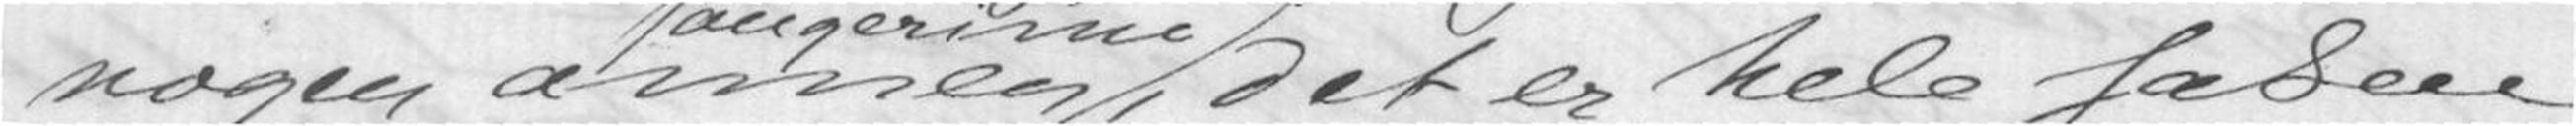nogen annen, det er hele saken
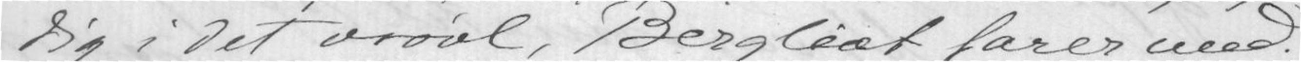dig i det vrøvl, Bergliot farer med.
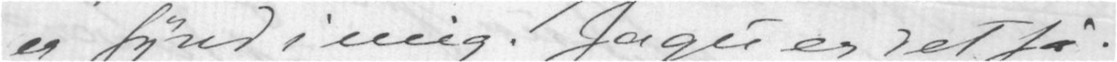er synd i mig. Jagu er det så.
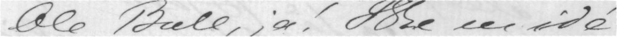Ole Bull, ja! Ikke en idé
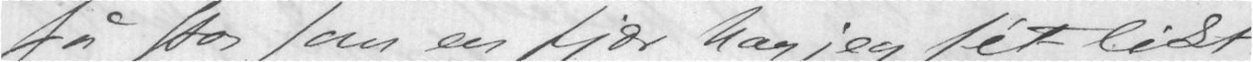så stor som en fjær har jeg set likt
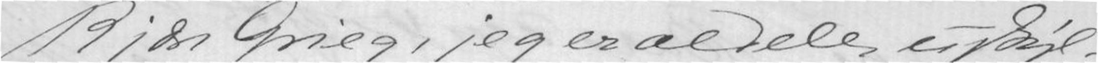Kjære Grieg, jeg er aldeles uskyl-
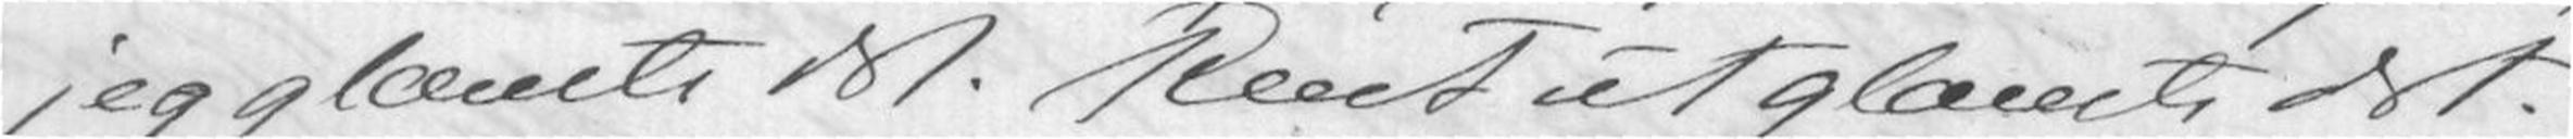jeg glæmte det. Rent ut glæmte det.
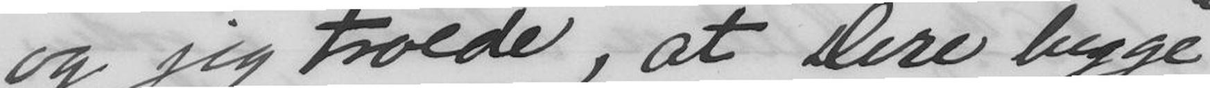og jeg troede, at Dere begge
